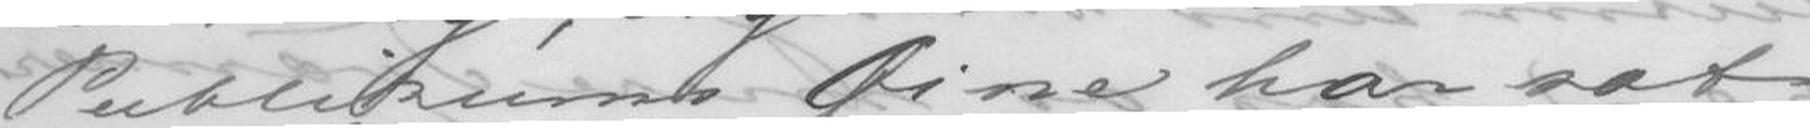Publikums Øine har sat
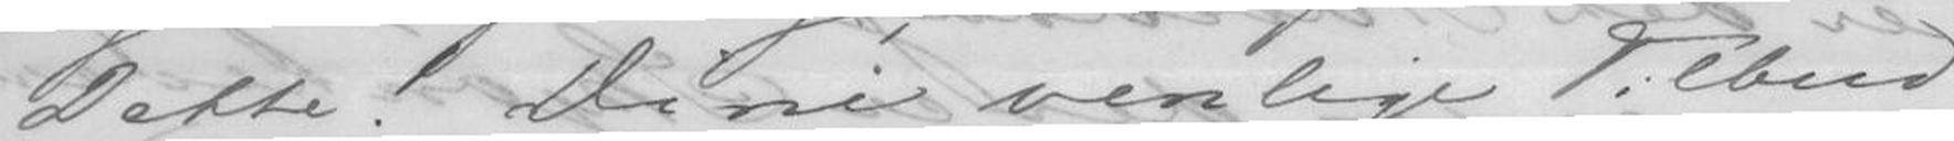Dette! Dine venlige Tilbud
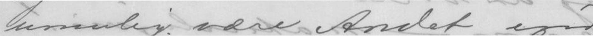umulig være Andet end
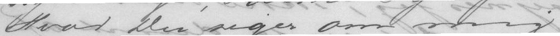Hvad Du siger om mig
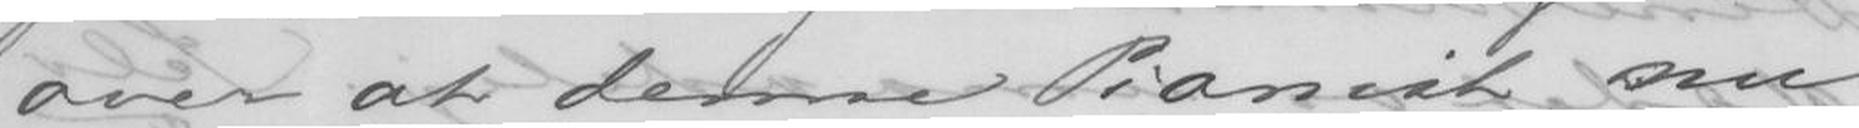over at denne Pianist nu
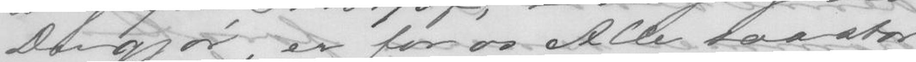Du gjør, er for os Alle saa stor
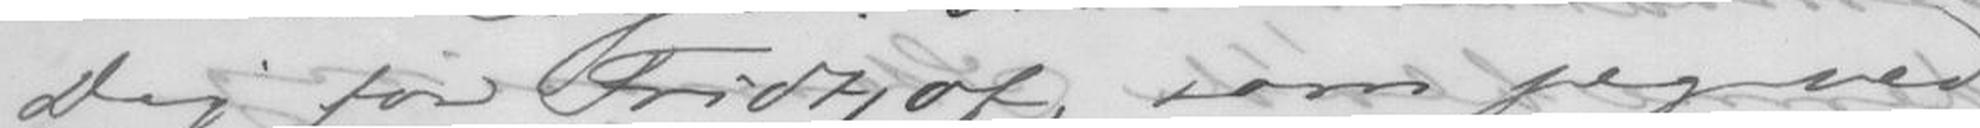Dig for Fridtjof, som jeg ved
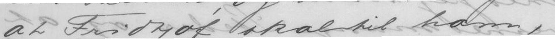at Fridtjof skal til ham,
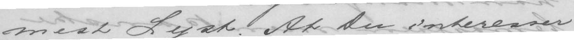mest Lyst. At Du interesser
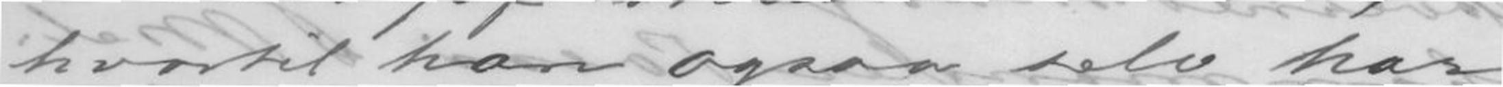hvortil han ogsaa selv har
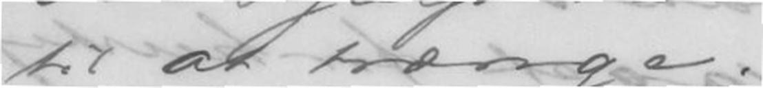til at trænge.
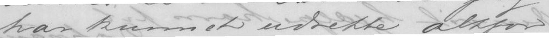har kunnet udrette altfor
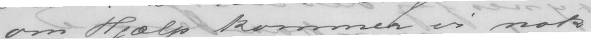om Hjælp kommer vi nok
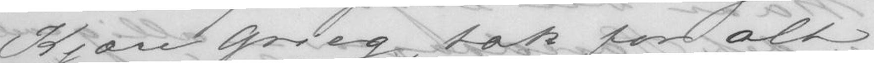Kjære Grieg, tak for alt
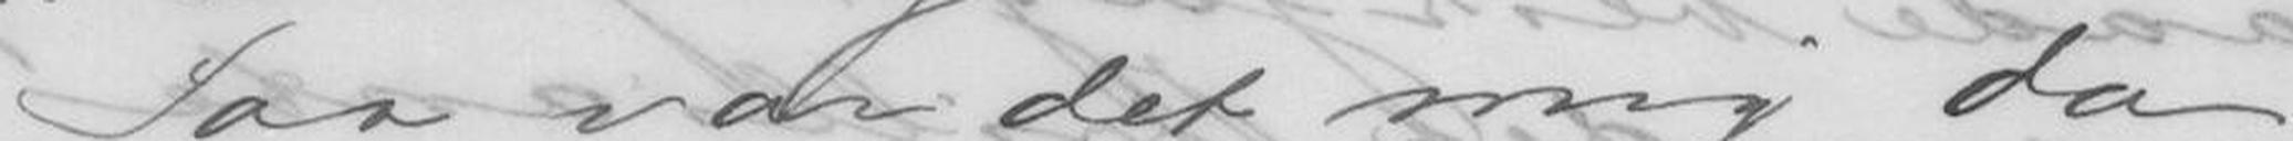Saa var det mig da
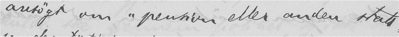ansøgt om "pension eller anden stats-
